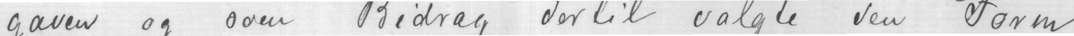gaven og som Bidrag dertil valgte den Form
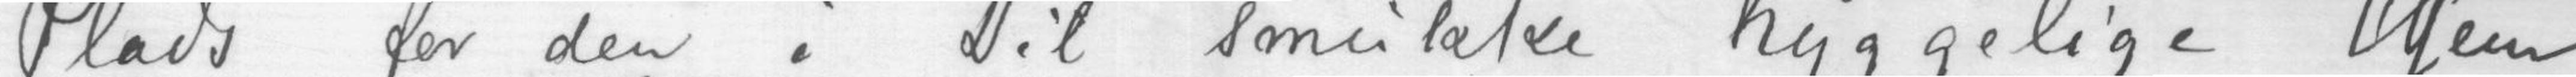Plads for den i Dit smukke hyggelige Hjem
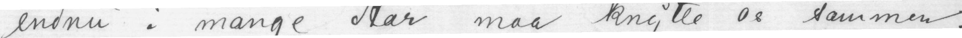endnu i mange Aar maa knytte os sammen.
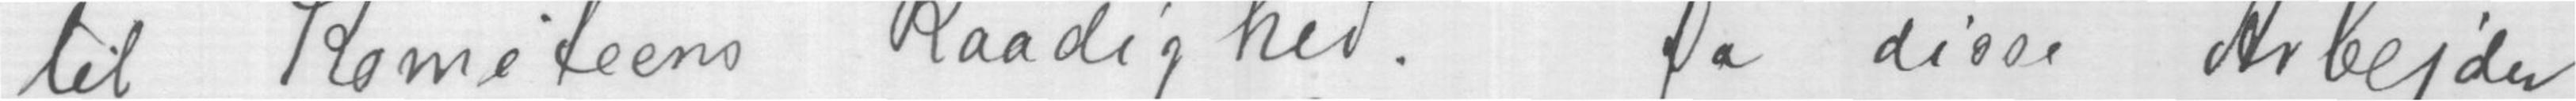til Komiteens Raadighed. Da disse Arbejder
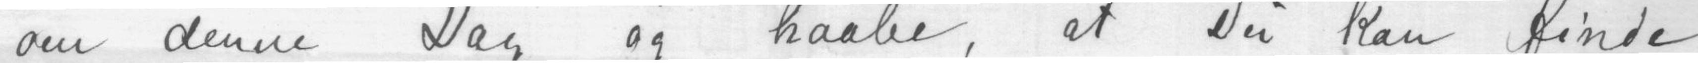om denne Dag og haabe, at Du kan finde
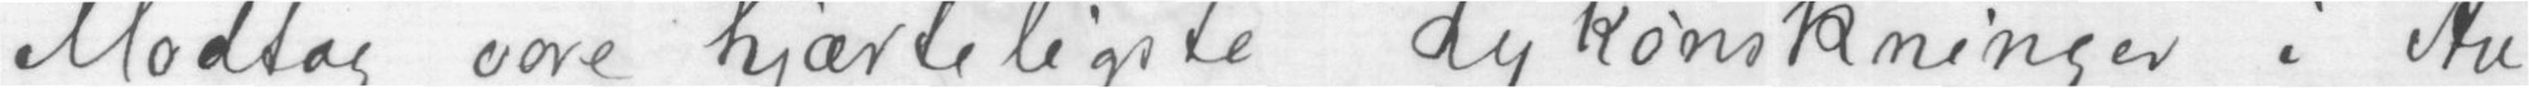Modtag vore hjærteligste Lykønskninger i An-
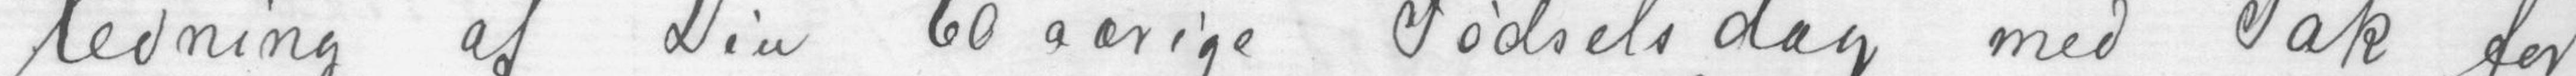ledning af Din 60 særige Fødselsdag med Tak for
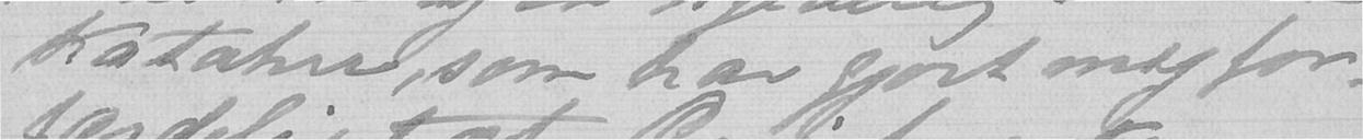katahrr, som har gjort mig for-
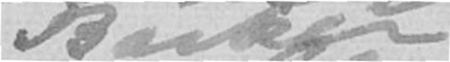Backer
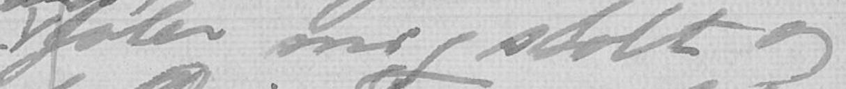føler mig stolt og
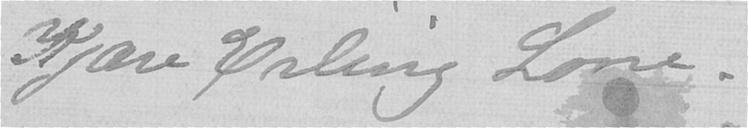Kjære Erling Lone.
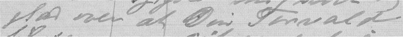glad over at Din Torvald
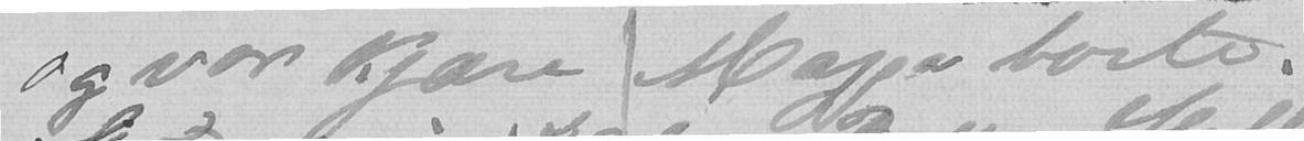og vor Kjære Magga borte.
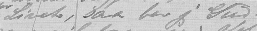Livet, saa ber jeg Gud -
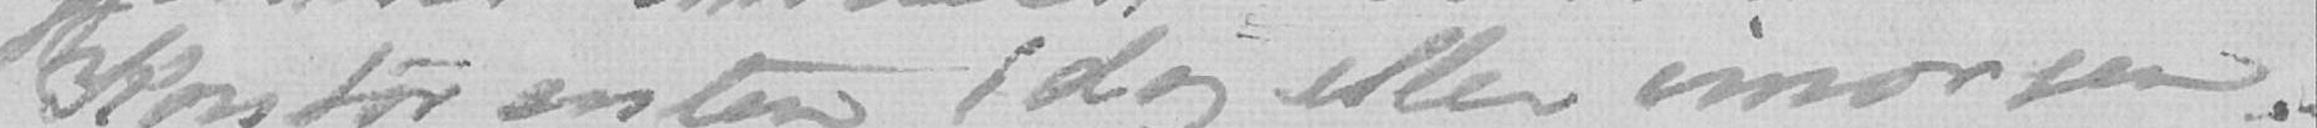Kontor enten idag eller imorgen.
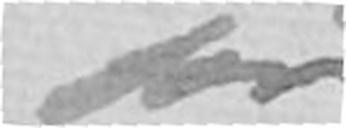for
